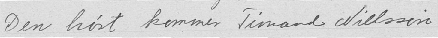Den høst kommer Timand Nielssøn
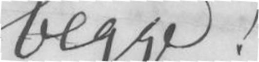begge!
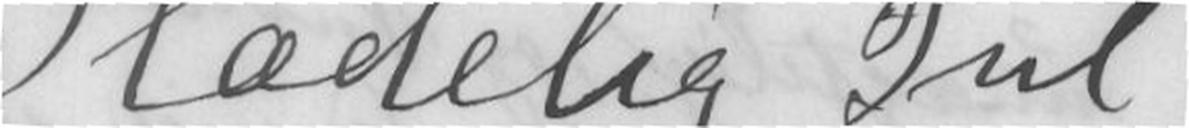Glædelig Jul!
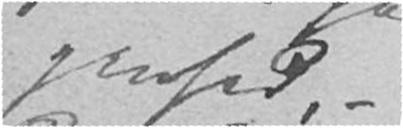genhed, -
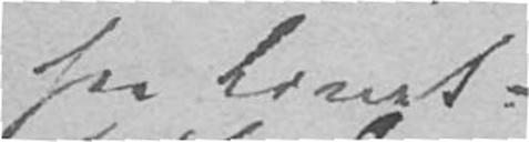fra Livet
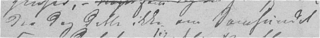der dog dette ikke om Samfundet
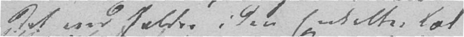det end falder i den Enkeltes Lod
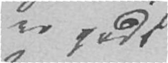er godt
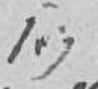sig
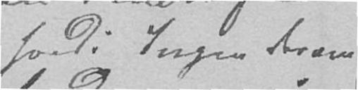fordi Ingen derom
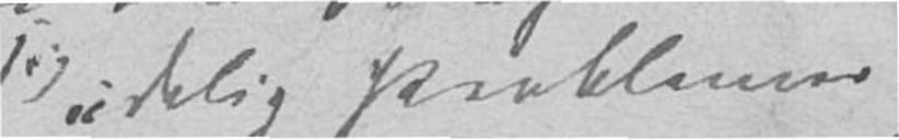idelig Problemer
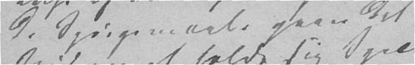de Spørgsmaale gaaer det
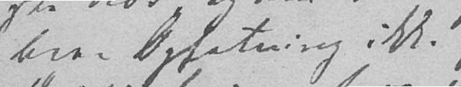bere Opfatning ikke
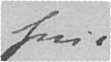hvis
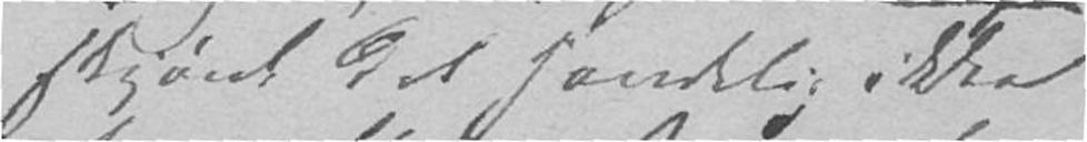,skjønt det sandelig ikke
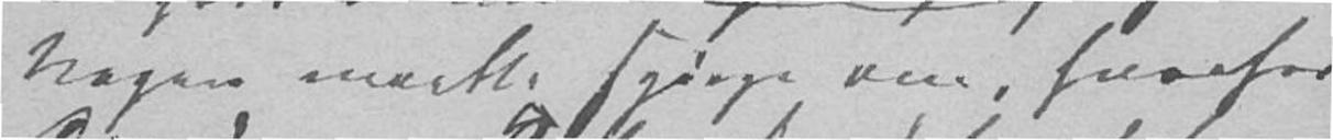Nogen maatte Spørge om, hvorfor
